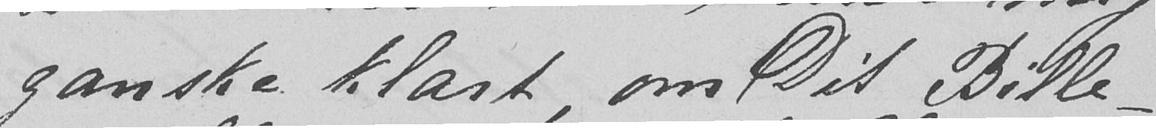ganske klart, om Dit Bille-
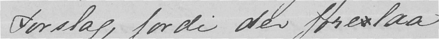Forslag, fordi der foreslaa
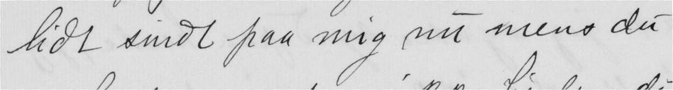lidt sendt paa mig nu mens du
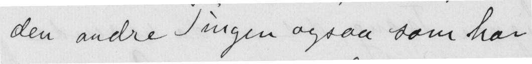den andre Tingen ogsaa som har
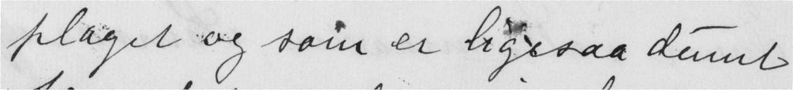plaget og som er ligesaa dumt
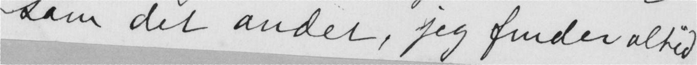som det andet, jeg finder altid
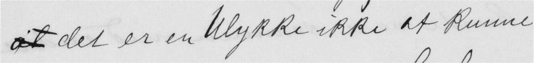at det er en Ulykke ikke at kunne
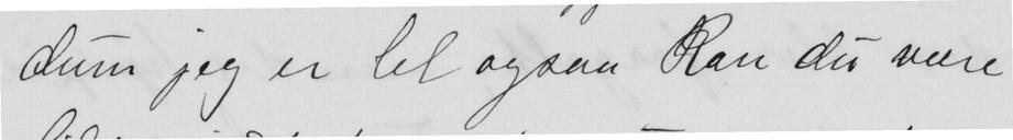dum jeg er lel ogsaa Kan du være
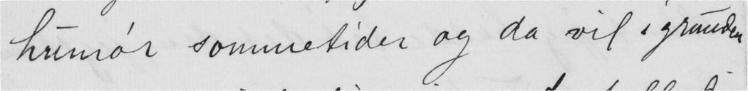Humør sommetider og da vil i grunden
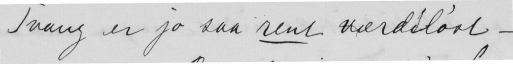Trang er jo saa sent værdiløst -
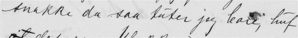snakke da saa tuter jeg bare, huf
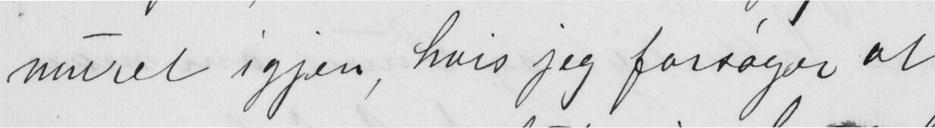muret igjen, hvis jeg forsøger at
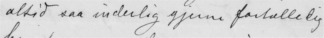altid saa inderlig gjerne fortælle dig
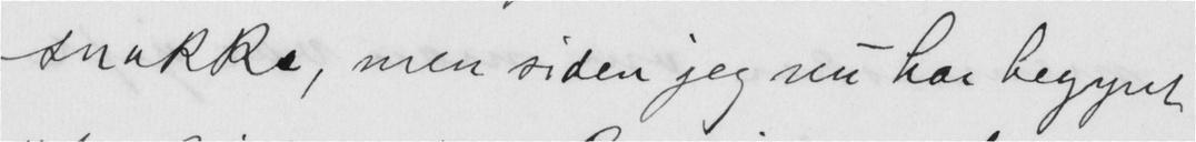snakke, men siden jeg nu har begynt
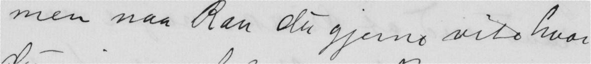men naa Kan du gjerne vide hvor
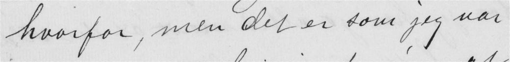hvorfor, men det er som jeg var
